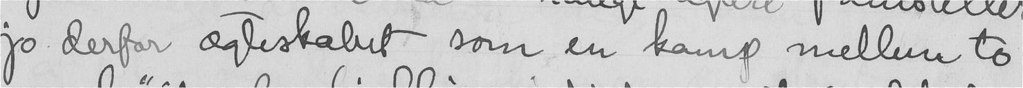jo derfor ægteskabet som en kamp mellem to
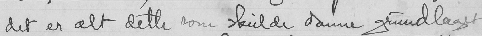det er alt dette som skulde danne grundlaget
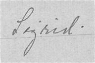Sigrid.
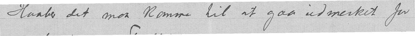Haaber det maa komme til at gaa udmerket for
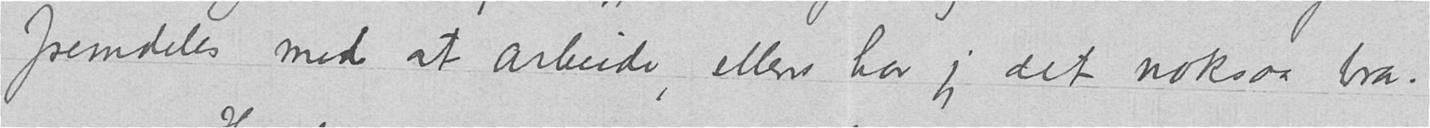fremdeles med at arbeide, ellers har jeg det noksaa bra.
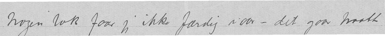Nogen bok faar jeg ikke færdig iaar – det gaar traatt
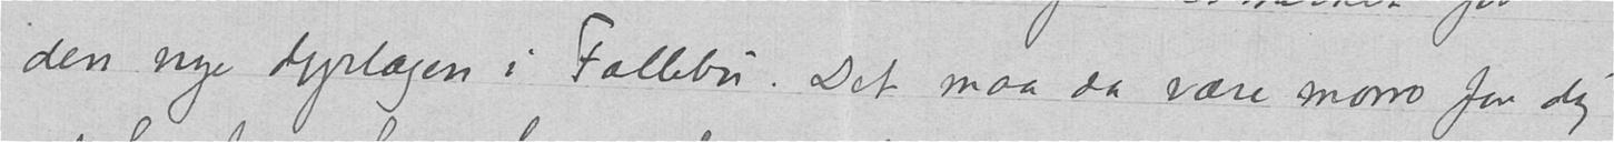den nye dyrlægen i Follebu. Det maa da være morro for dig
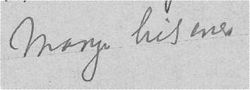Mange hilsener
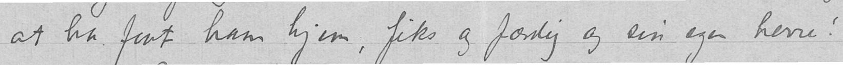at ha faat ham hjem, fiks og færdig og sin egen herre!
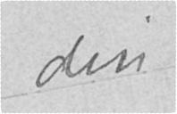din
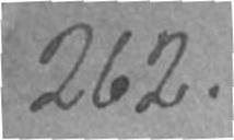262.
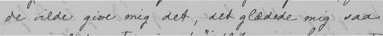de vilde give mig det, det glædede mig saa
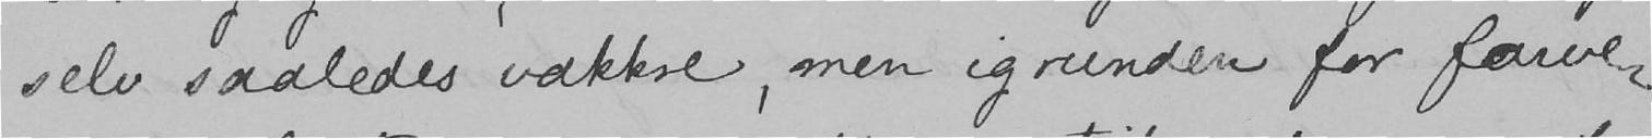selv saaledes vakkre, men igrunden for farve
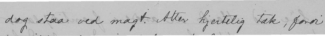dog staa ved magt. Atter hjertelig tak, fordi
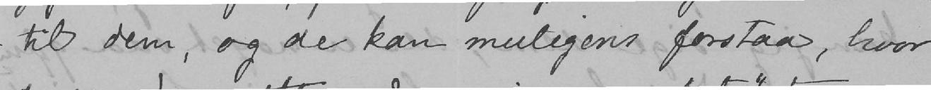til dem, og de kan muligens forstaa, hvor
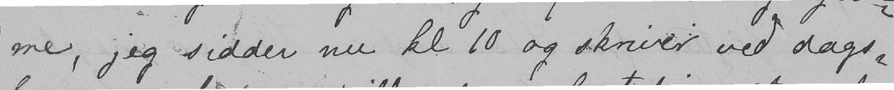me, jeg sidder nu kl 10 og skriver ved dags-
